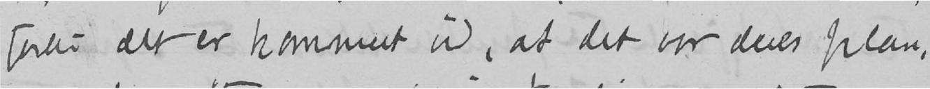fordi alt er kommet ud, at det var deres plan,
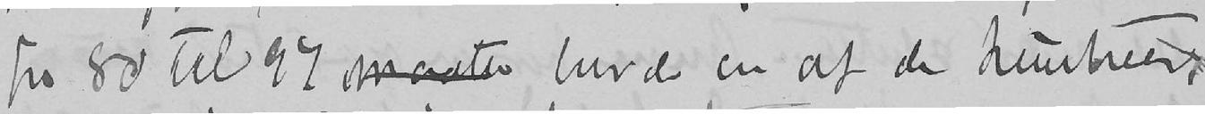fra 80 til 97 maatte burde en af de kunstnere,
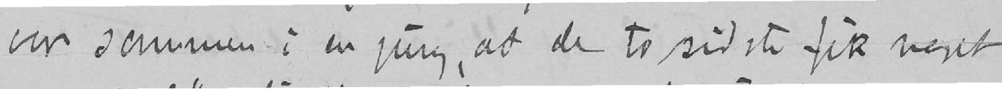var sammen i en jury, at de to sidste fik noget
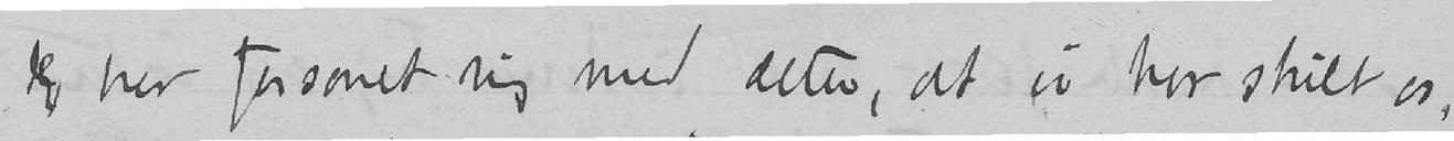Jeg har forsonet mig med dette, at vi har skilt os,
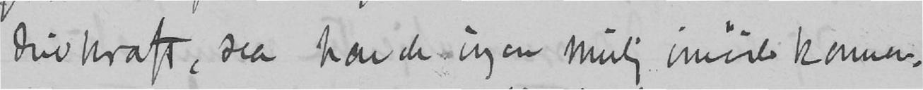drivkraft, saa havde ingen mulig imødekommen-
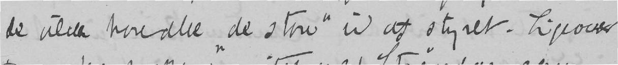de vilde have alle «de store» ud af styret. Ligeover
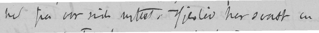hed fra vor side nyttet. Hjerløv har svart en,
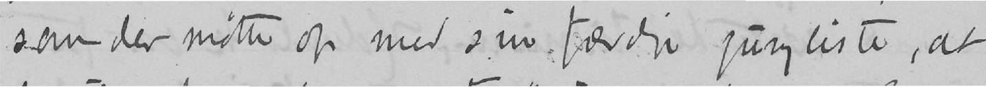som der møtte op med sin færdige juryliste, at
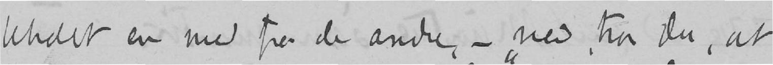beholdt en med fra de andre, – «nei, tror du, at
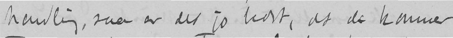handling, saa er det jo bedst, at de kommer
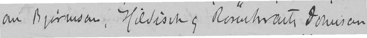om Bjørnson, Hildisch og Rosenkrantz Johnsen
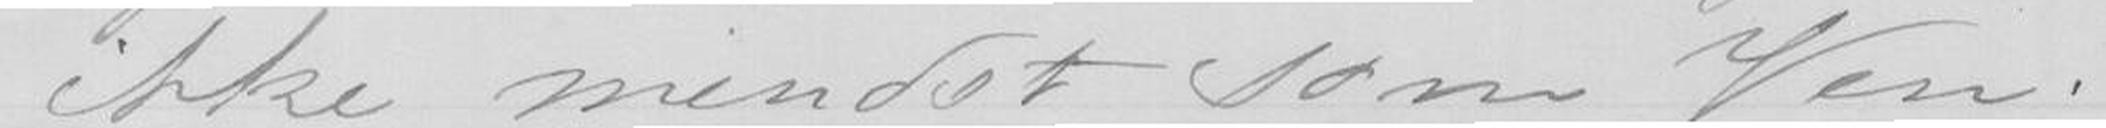ikke mindst som Ven
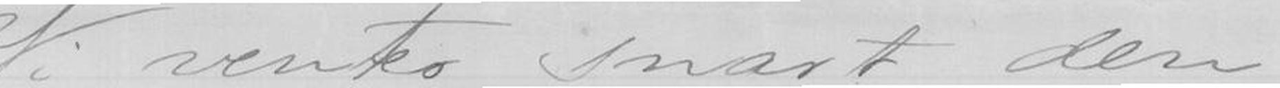Vi venter snart den
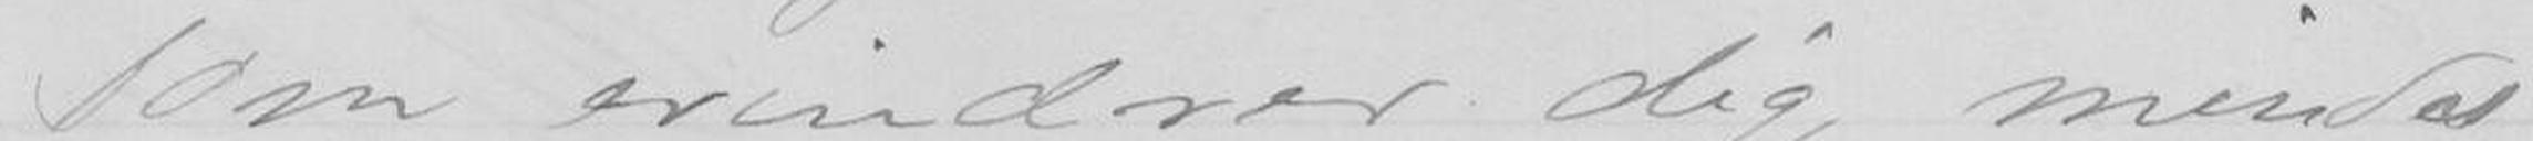som erindrer dig, mindes
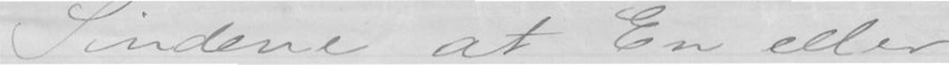Sindene at En eller
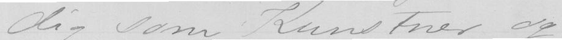dig som Kunstner og
